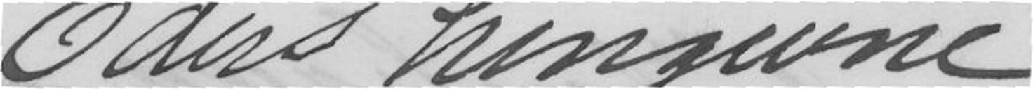Eders hengivne
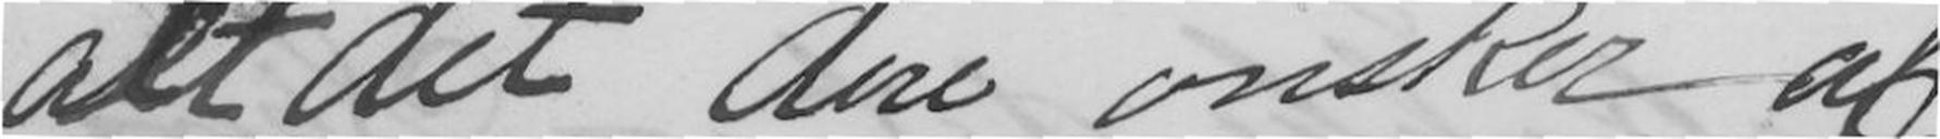alt det dere ønsker af
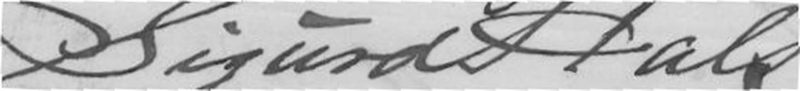Sigurd Hals.
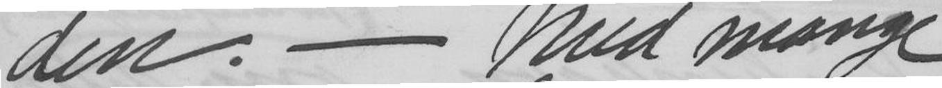den. - Med mange
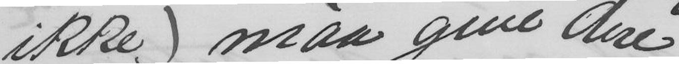ikke) maa give dere
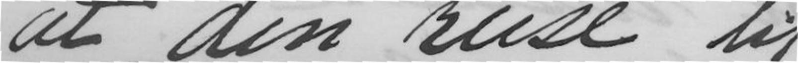at den reise til
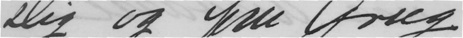Dig og fru Grieg
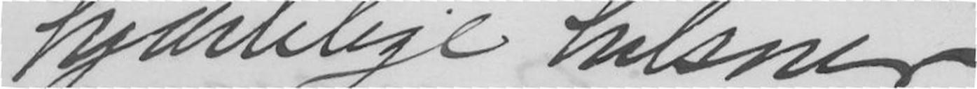hjærtelige hilsner
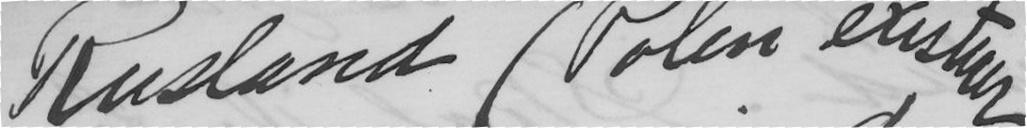Rusland (Polen existerer
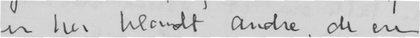er her blandt andre, de er
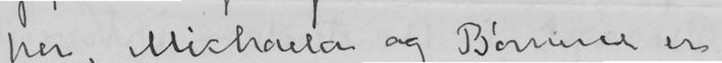her, Michaela og Børnene er
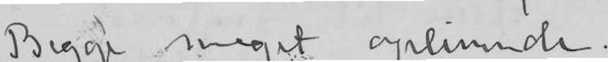Begge meget oplivende
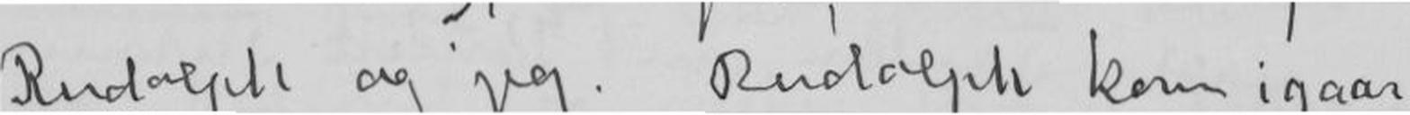Rudolph og jeg. Rudolph kom igaar.
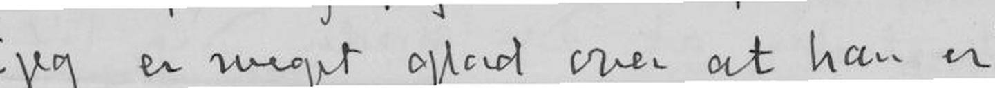jeg er meget glad over at han er
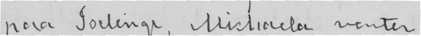paa Soelinge Michaela venter
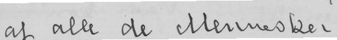af alle de Mennesker.
